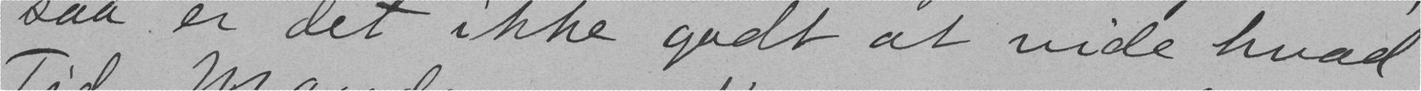saa er det ikke godt at vide hvad
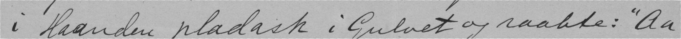i Haanden pladask i Gulvet og raabte: "Aa
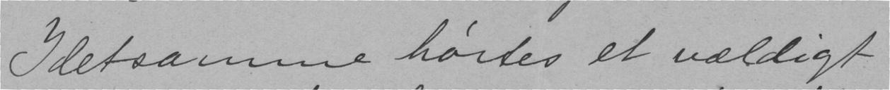Idetsamme hørdes et vældigt
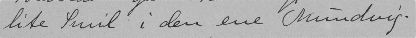lite Smil i den ene Mundvig.
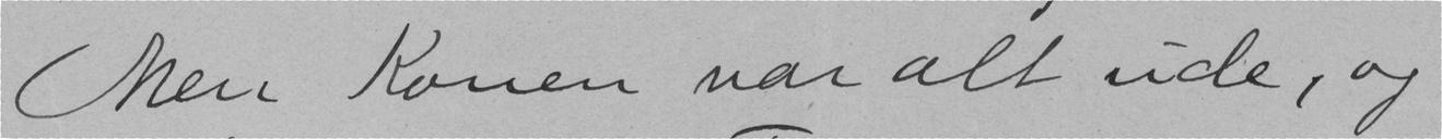Men Konen var alt ude, og
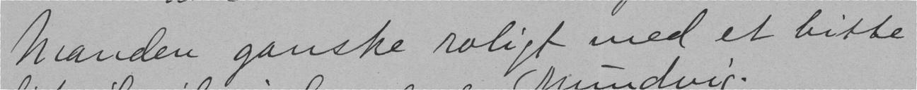Manden ganske roligt med et bitte
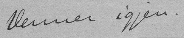Venner igjen.
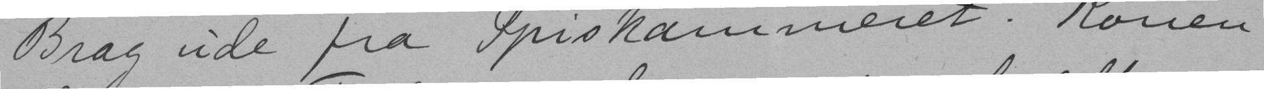Brag ude fra Spiskammeret. Konen
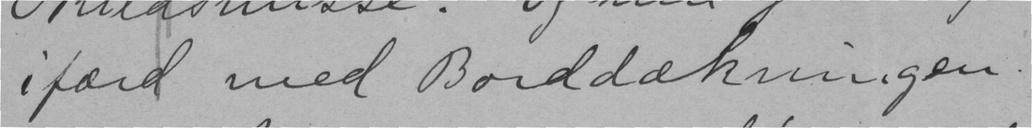ifærd med Borddækningen.
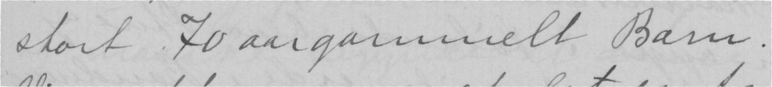stort 70 aargammelt Barn.
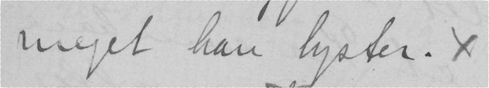meget han lyster. x
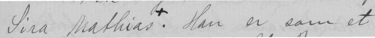Fira Mathias. Han er som et
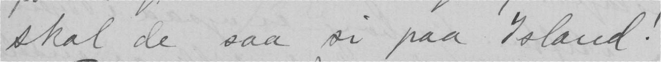skal de saa si paa Island!
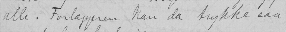alle. Forlæggeren kan da trykke saa
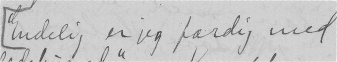Endelig er jeg færdig med
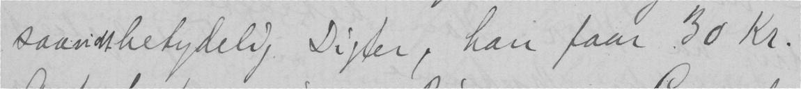saavidtbetydelig Digter, han faar 30 Kr.
